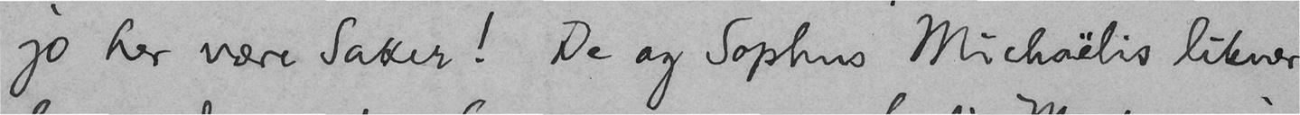jo her være Saker! De og Sophus Michaëlis likner
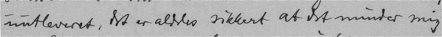uutleveret, det er aldeles sikkert at det minder mig
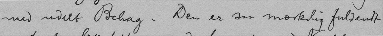med udelt Behag. Den er saa mærkelig fuldendt
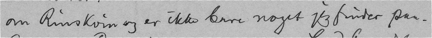om Rinskvin og er ikke bare noget jeg finder paa.
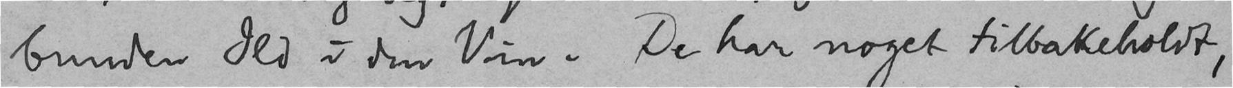bunden Ild i den Vin. De har noget tilbakeholdt,
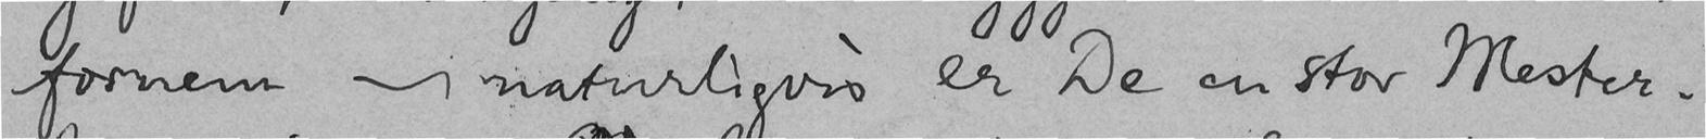fornem - naturligvis er De en stor Mester.
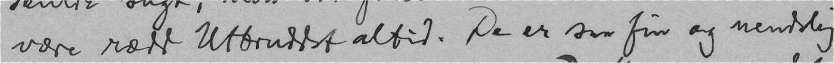være rædd Utbruddet altid. De er saa fin og uendlig
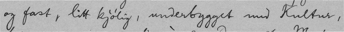og fast, litt kjølig, underbygget med Kultur,
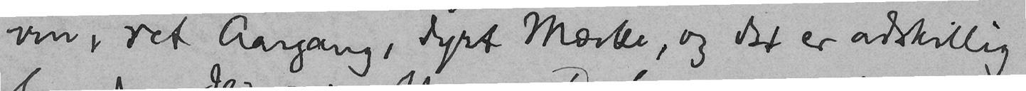vin, ret Aargang, dyrt Mærke, og det er adskillig
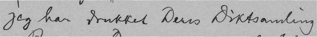jeg har drukket Deres Diktsamling
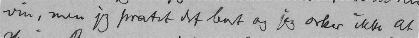vin, men jeg pratet det bort og jeg orker ikke at
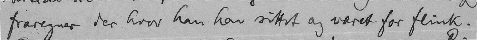fraregner der hvor han har sittet og været for flink.
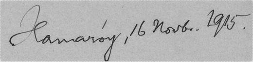Hamarøy, 16 Novbr. 1915.
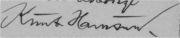Knut Hamsun.
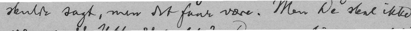skulde sagt, men det faar være. Men De skal ikke
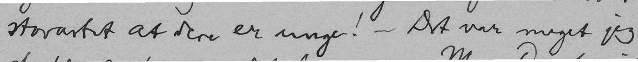storartet at dere er unge! - Det var meget jeg
